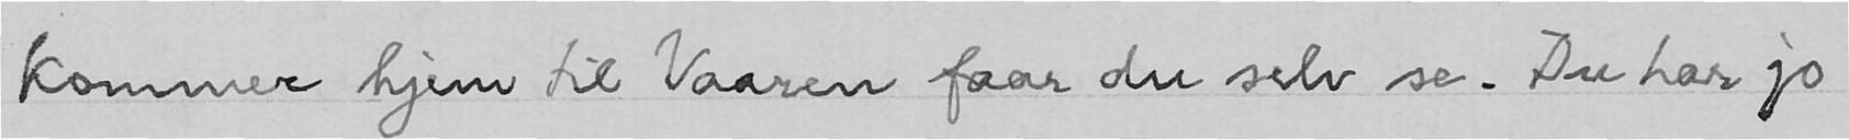kommer hjem til Vaaren faar du selv se. Du har jo
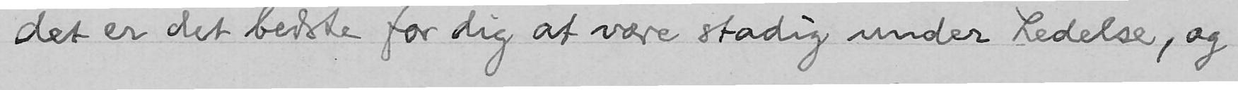det er det bedste for dig at være stadig under Ledelse, og
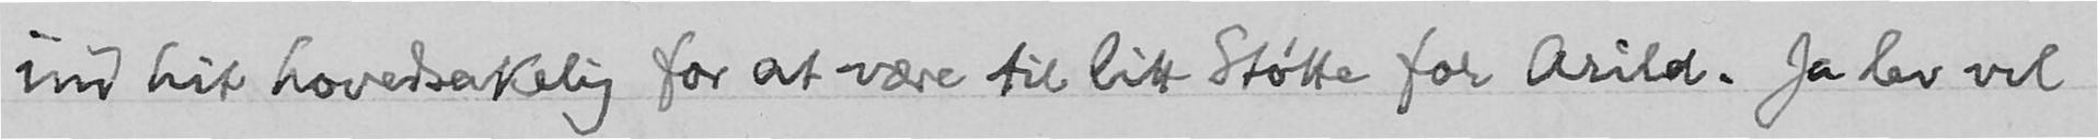ind hit hovedsakelig for at være til litt Støtte for Arild. Ja lev vel
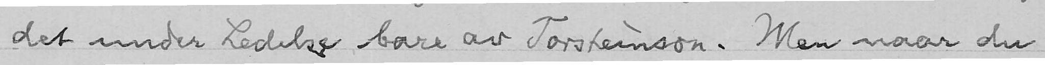det under Ledelse bare av Torsteinson. Men naar du
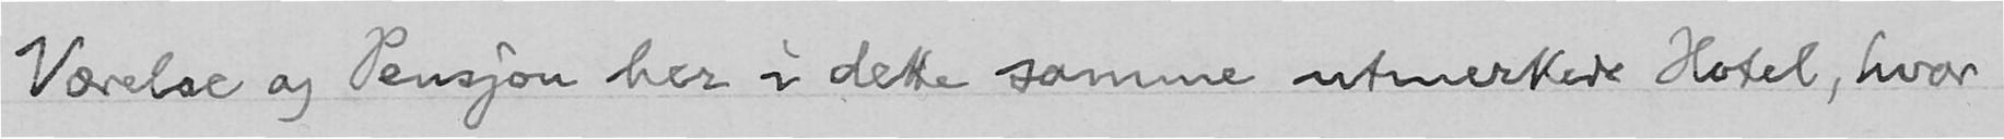Værelse og Pensjon her i dette samme utmerkede Hotel, hvor
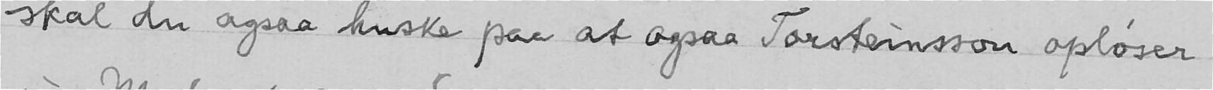skal du ogsaa huske paa at ogsaa Torsteinsson opløser
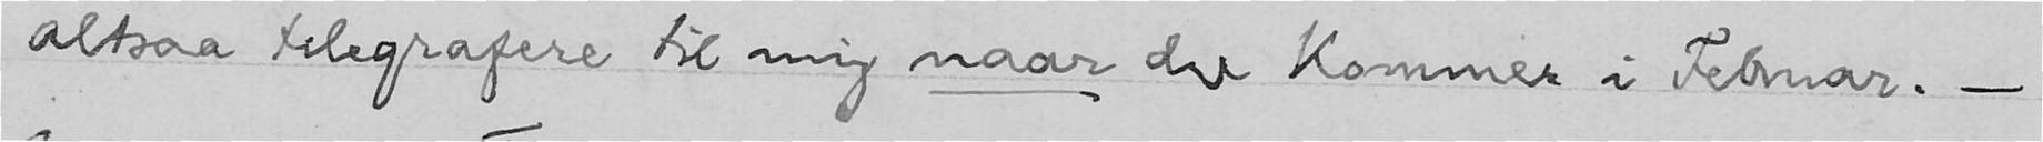altsaa telegrafere til mig naar du kommer i Februar. -
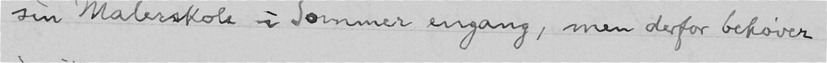sin Malerskole i Sommer engang, men derfor behøver
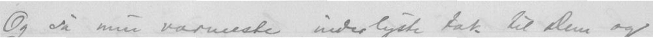Og så min varmeste, inderligste tak til Dem og
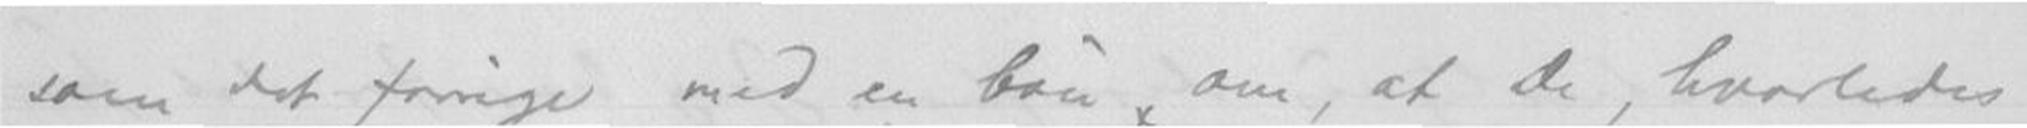som det forige med bøn om, at De, hvorledes
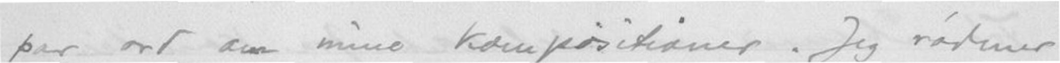par ord om mine kompositioner. Jeg rødmer
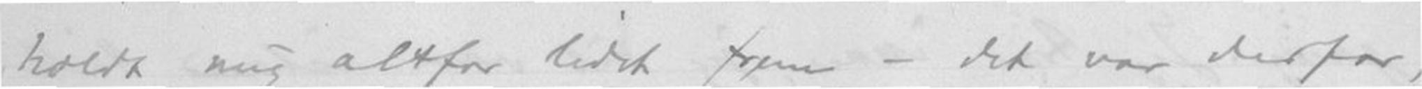holdt mig altfor lidet frem - det var derfor,
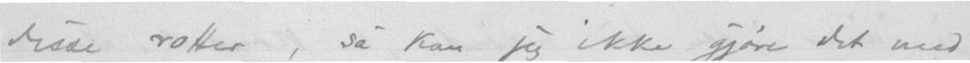disse rotter, så kan jeg ikke gjøre det med
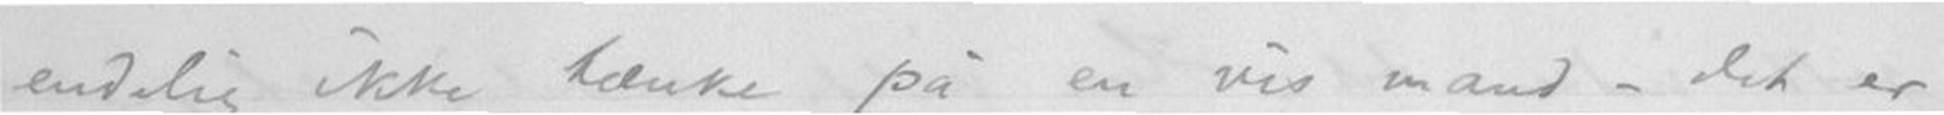endelig ikke tænke på en vis mand - det er
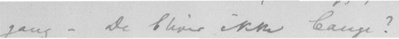gang - De bliver ikke bange?
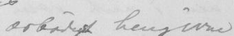ærbødigst hengivne
